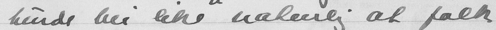burde bli like naturlig at folk
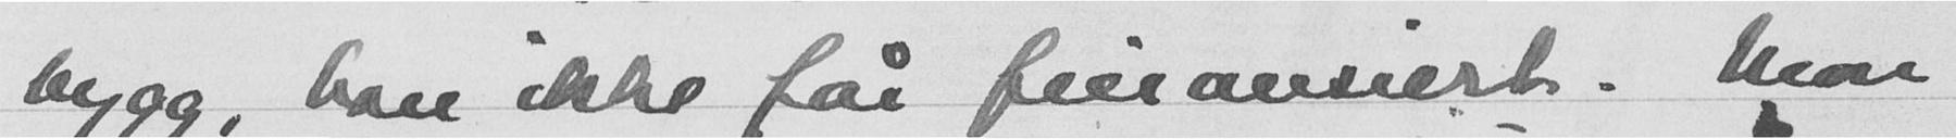bygg, han ikke får finansiert. Når
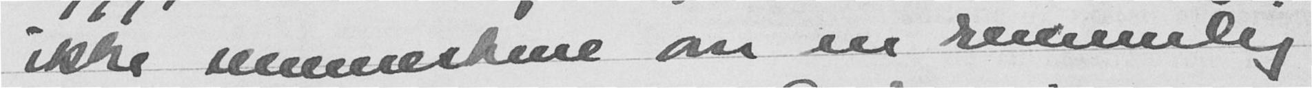ikke menneskene av en rummlig
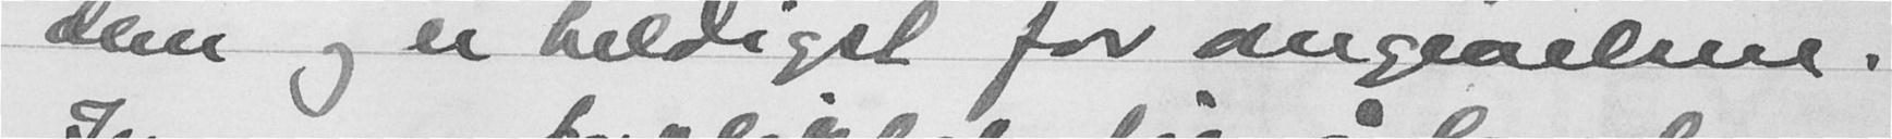dem og er heldigst for avgjørelsen.
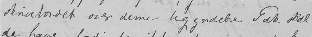skrivebordet over denne begyndelse. Tak skal
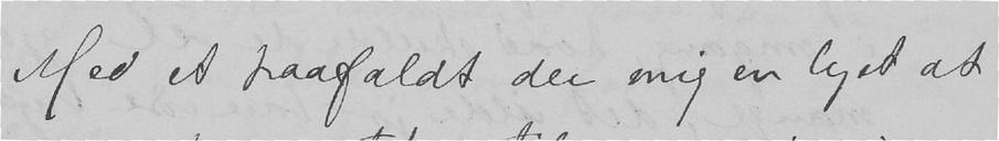Med et paafaldt der mig en lyst at
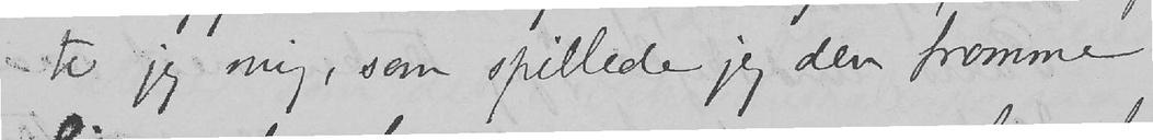te jeg mig, som spillede jeg den fromme
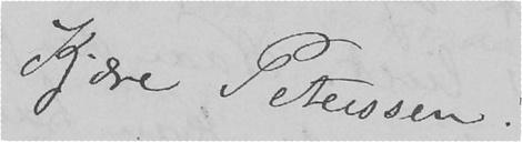Kjære Peterssen!
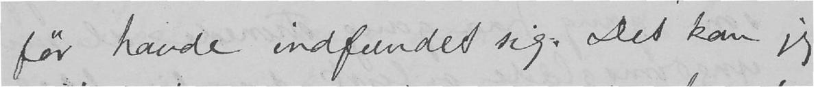før havde indfundet sig. Det kan jeg
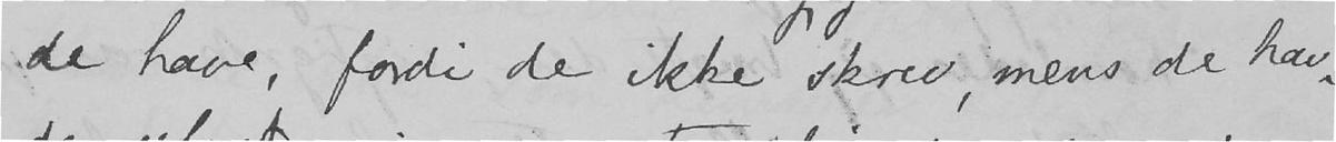de have, fordi de ikke skrev, mens de hav-
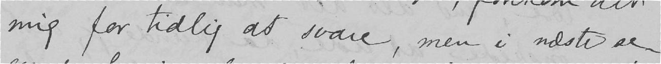mig for tidlig at svare, men i næste se-
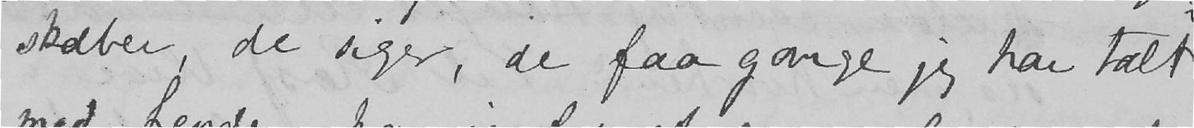skaber, de siger, de faa gange jeg har talt
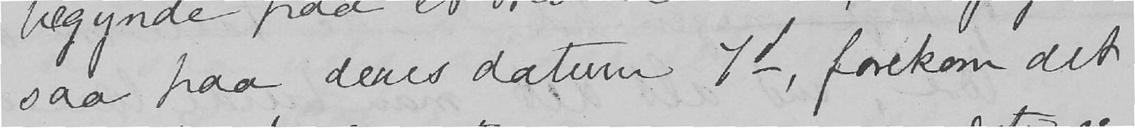saa paa deres datum 7d, forekom det
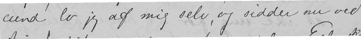cund lo jeg af mig selv, og sidder nu ved
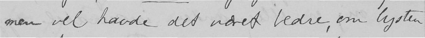men vel havde det været bedre, om lysten
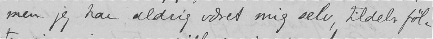men jeg har aldrig været mig selv, tildels føl-
